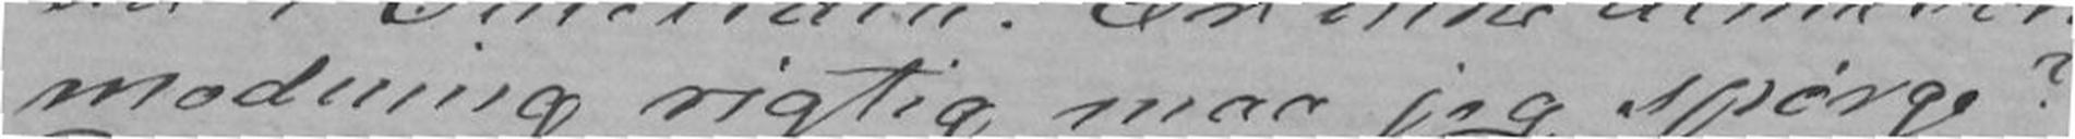modning rigtig maa jeg spørge?
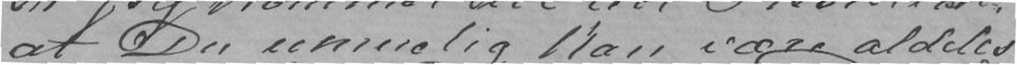at Du umuelig kan være aldeles
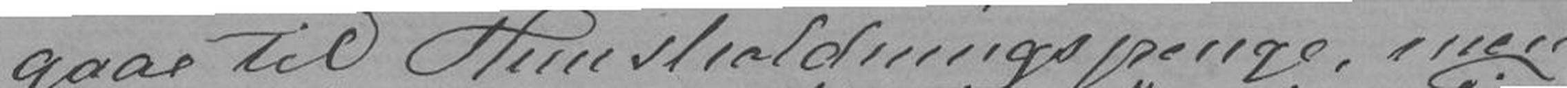gaae til Huusholdningspenge, men
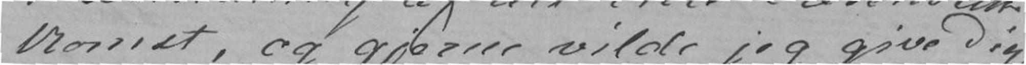komst, og gjerne vilde jeg give Dig
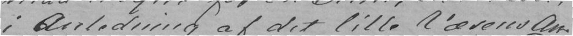i Anledning af det lille Væsens An-
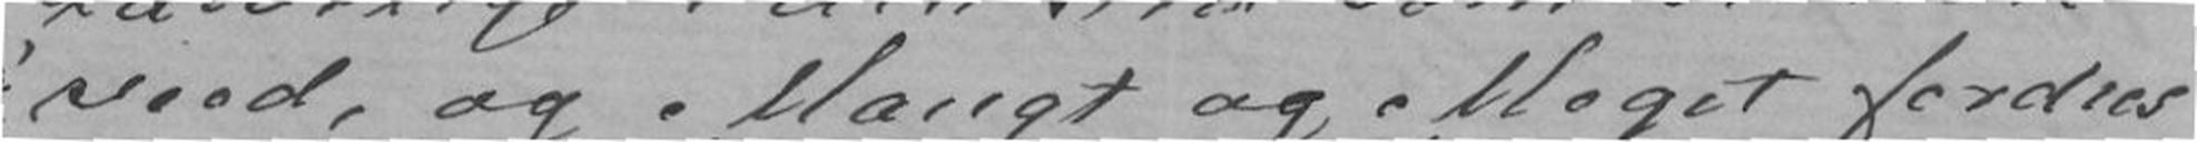veed, og Mangt og Meget fordres
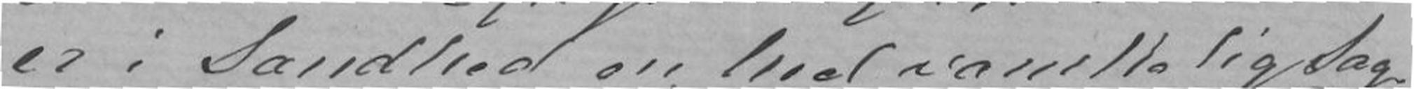er i Sandhed en heel vanskelig Sag.
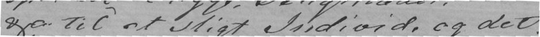ogc. til et sligt Individ, og det
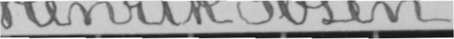Henrik Ibsen.
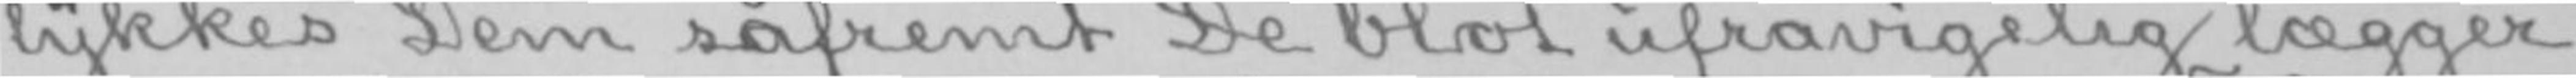lykkes Dem såfremt De blot ufravigelig lægger
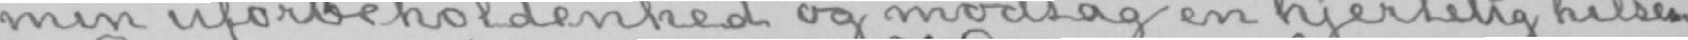min uforbeholdenhed og modtag en hjertelig hilsen
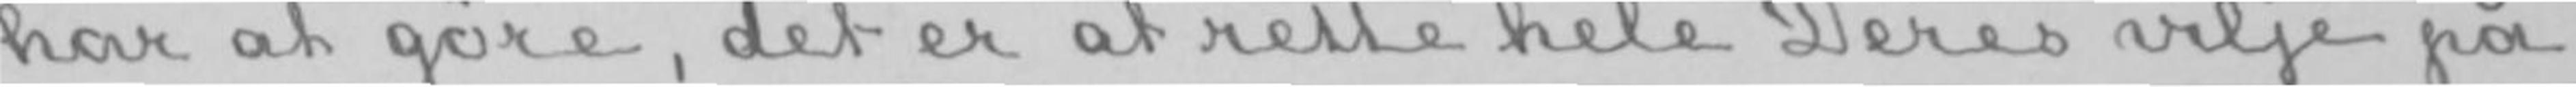har at gøre, det er at rette hele Deres vilje på
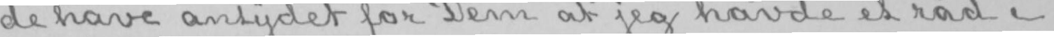de have antydet for Dem at jeg havde et råd i
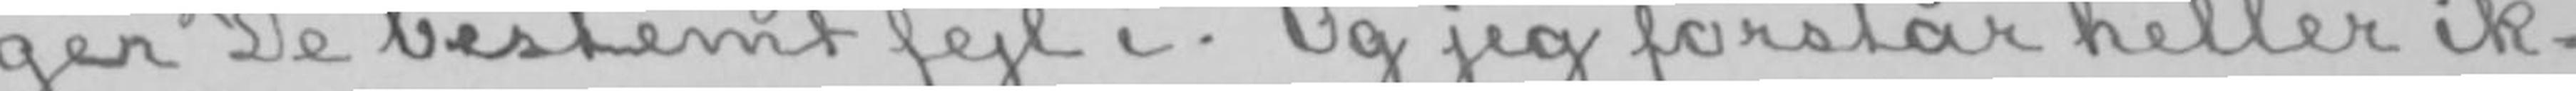ger De bestemt fejl i. Og jeg forstår heller ik-
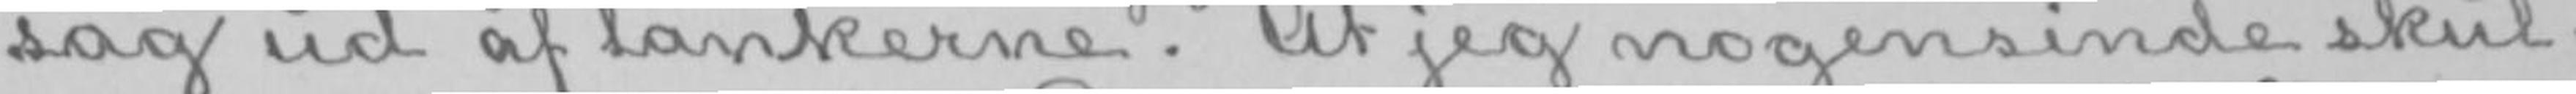sag ud af tankerne. At jeg nogensinde skul-
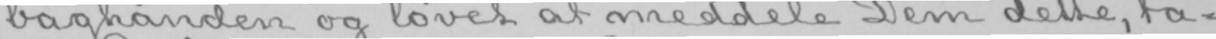baghånden og lovet at meddele Dem dette, ta-
